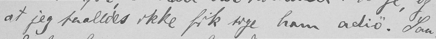at jeg saaledes ikke fik sige ham adiø. Saa-
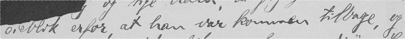øieblik erfor, at han var kommen tilbage, og
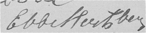Ebbe Hertzberg.
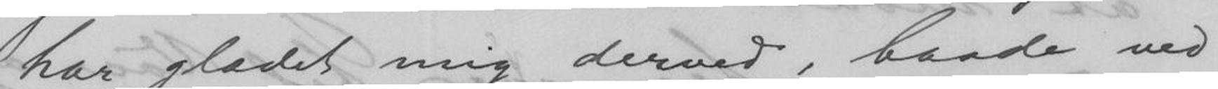har glædet mig derved, baade ved
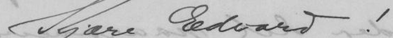Kjære Edvard!
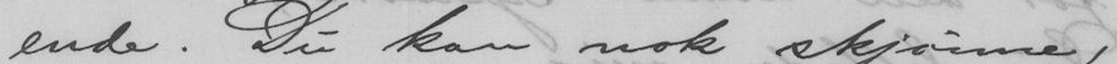ende. Du kan nok skjønne,
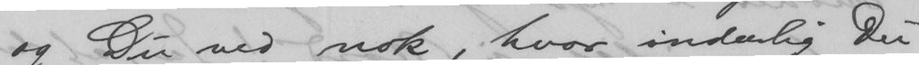og Du ved nok, hvor inderlig Du
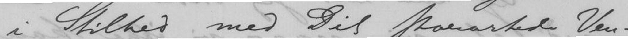i Stilhed med Dit storartede Ven-
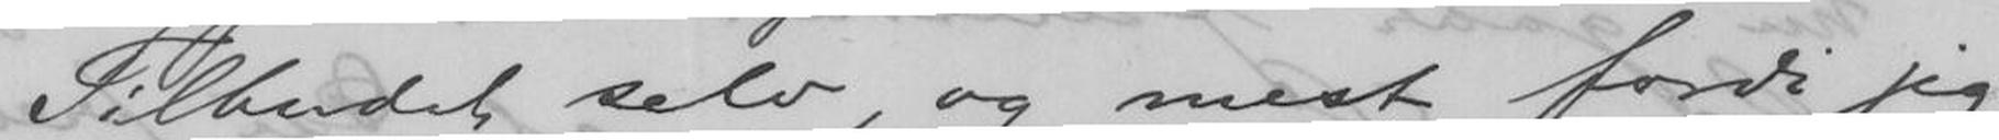Tilbudet selv, og mest fordi jeg
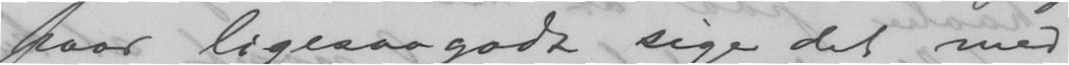faar ligesaagodt sige det med
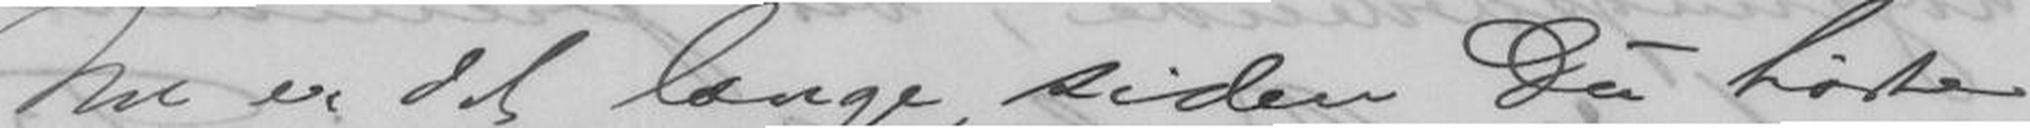Nu er det længe siden Du hørte
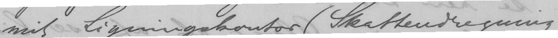mit Ligningskontor (Skatteudregning
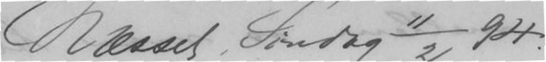Næsset Søndag 11/2 94.
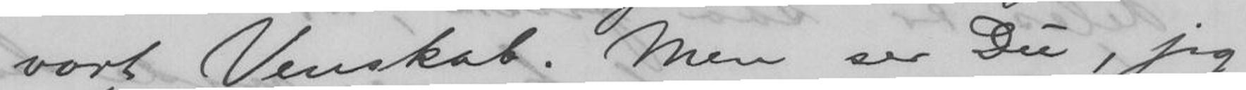vort Venskab. Men ser Du, jeg
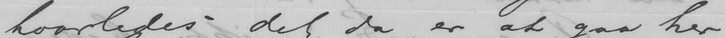hvorledes det da er at gaa her
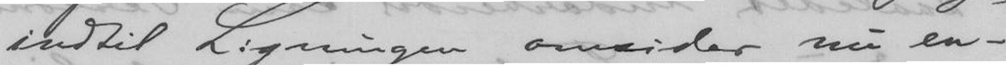indtil Ligningen omsider nu en-
